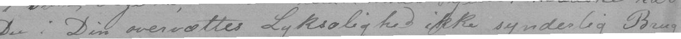Du i Din overvættes Lyksalihed ikke synderlig Brug
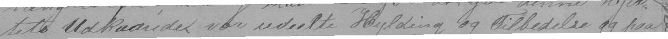tets Udkaarede vor udeelte Hyldning og Tilbedelse og paa
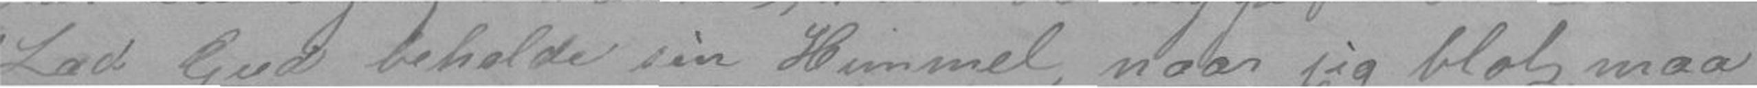"Lad Gud beholde sin Himmel, naar jeg blot maa
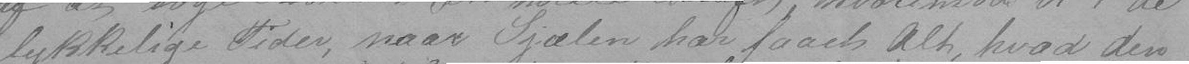lykkelige Tider, naar Skjælen har faaet Alt, hvad den
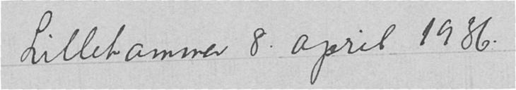Lillehammer 8. april 1936.
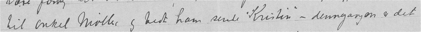til onkel Møller og bedt ham sende "Kristin" – dennegangen er det
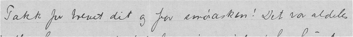Takk for brevet dit og for smørasken! Det var aldeles
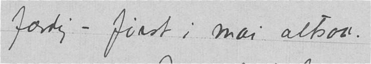færdig – først i mai altsaa.
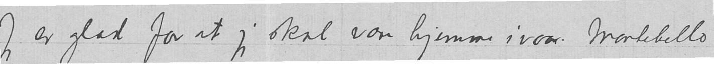Jeg er glad for at jeg skal være hjemme ivaar. Montebello
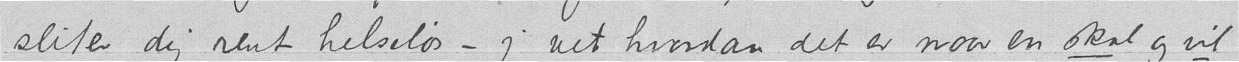sliter dig rent helseløs – jeg vet hvordan det er naar en skal og vil
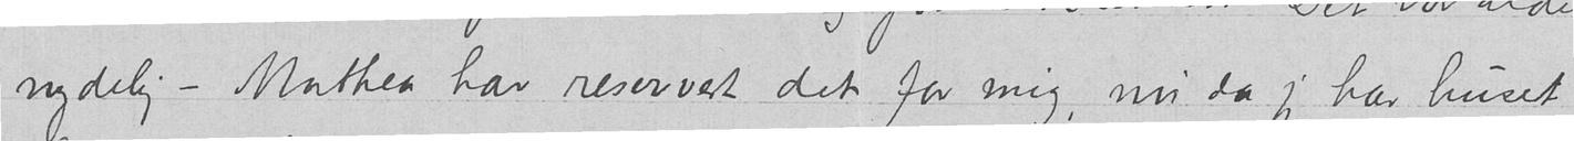nydelig - Mathea har reservert det for mig, nu da jeg har huset
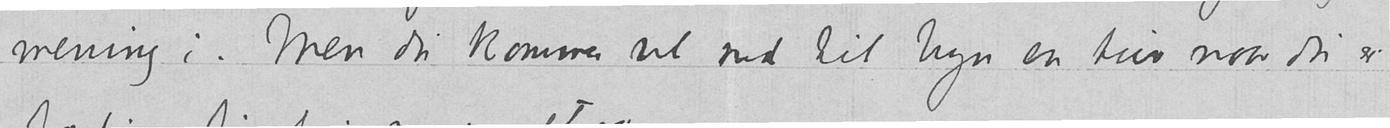mening i. Men du kommer vel ned til byn en tur naar du er
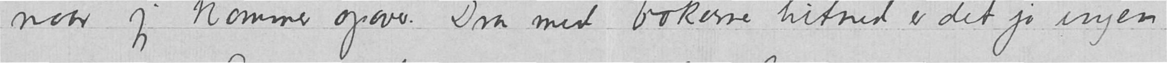naar jeg kommer opover. Dra med bøkerne hitned er det jo ingen
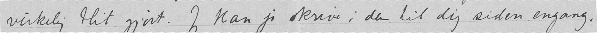virkelig blit gjort. Jeg kan jo skrive i den til dig siden engang,
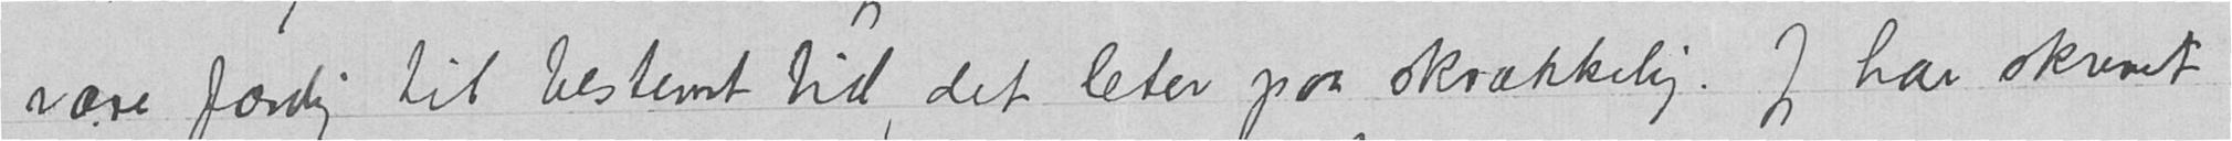være færdig til bestemt tid, det leter paa skrækkelig. Jeg har skrevet
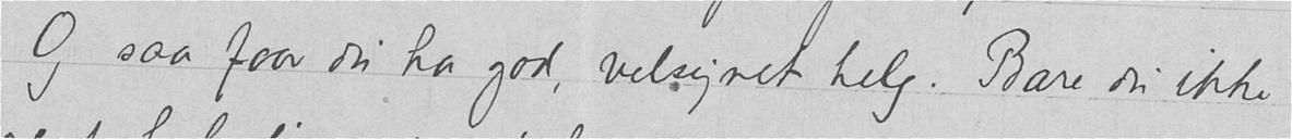Og saa faar du ha god, velsignet helg. Bare du ikke
